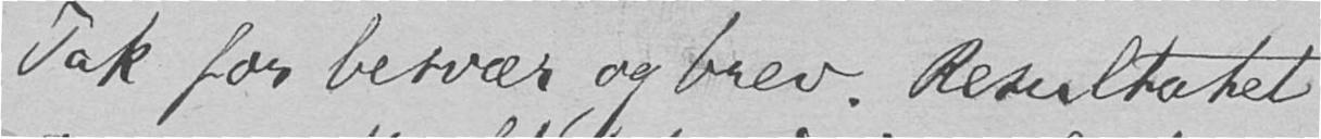Tak for besvær og brev. Resultatet
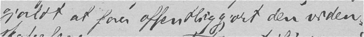gjaldt at faa offentliggjort den viden-
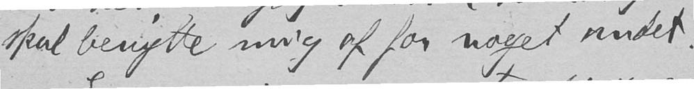skal benytte mig af for noget andet.
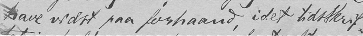have vidst paa forhaand, idet tidsskrif-
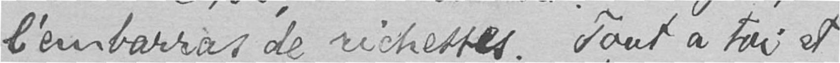l'embarras de richesses. Tout a toi et
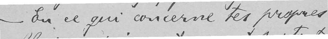– En ce qiu concerne tes propres
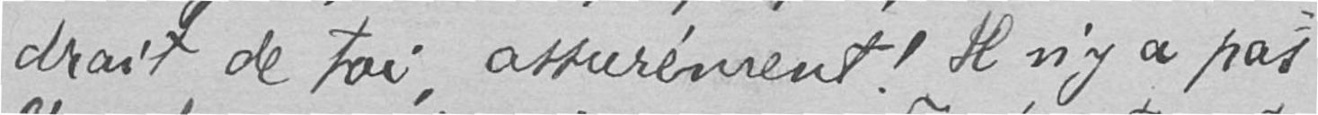drait de toi, assurément! H n'y a pas
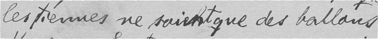les tiennes ne soint que des ballons
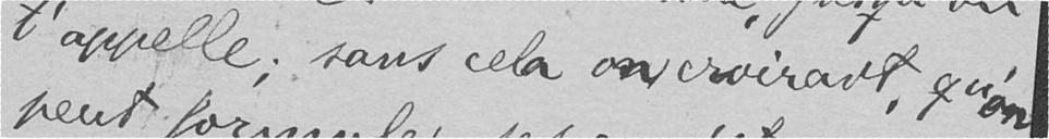t’appelle; sans cela on croirart, qu'on
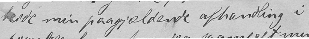beide min paagjældende afhandling i
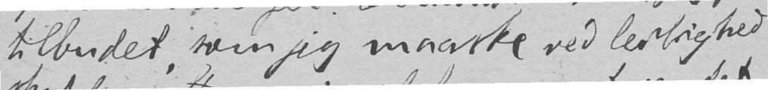tilbudet, som jeg maaske ved leilighed
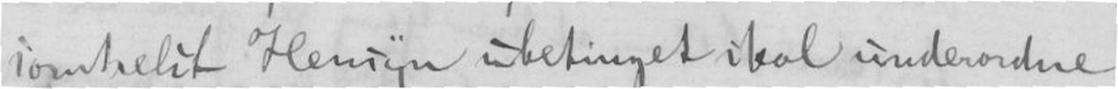somhelst Hensyn ubetinget skal underordne
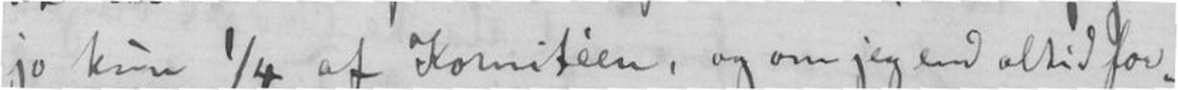jo kun 1/4 af Komitèen, og om jeg end altid for-
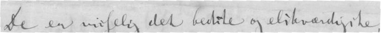De er visselig det bedste og elskværdigste,
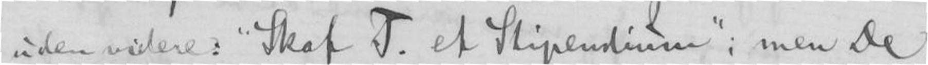uden videre: "Skaf T. et Stipendium"; men De
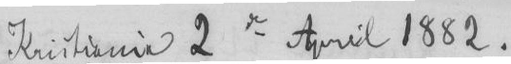Kristiania 2dre April 1882.
